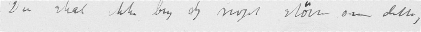Du skal ikke bry dig noget større om dette,
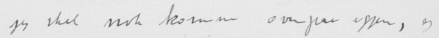jeg skal nok komme ovenpaa igjen, og
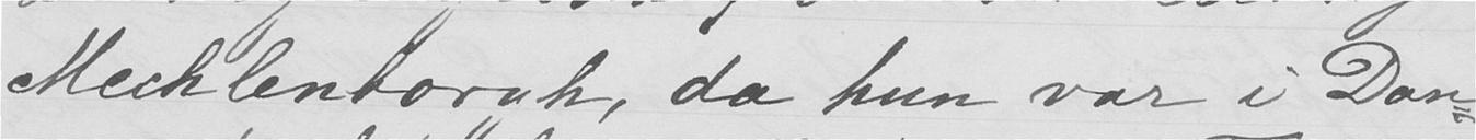Mechlenborgh, da hun var i Dan-
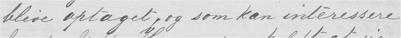blive optaget, og som kan interessere
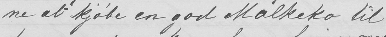ne at kjøbe en god Malkeko til
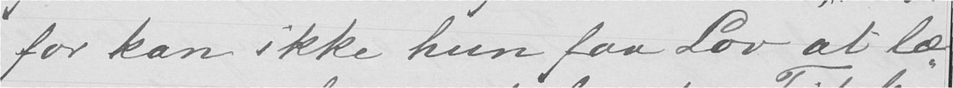for kan ikke hun faa Lov at læ-
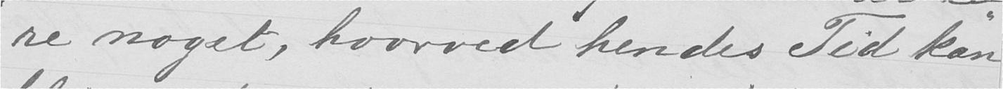re noget, hvorved hendes Tid kan
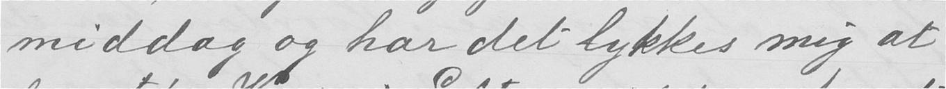middag og har det lykkes mig at
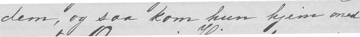dem, og saa kom hun hjem med
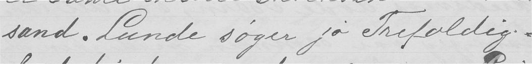sand. Lunde søger jo Trefoldig-
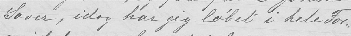Saver, idag har jeg løbet i hele For-
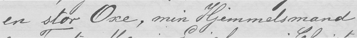en stor Oxe, min Hjemmelsmand
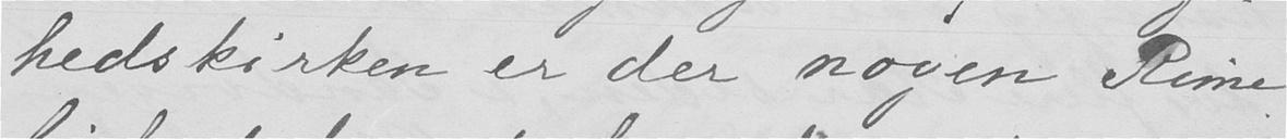hedskirken er der nogen Rime-
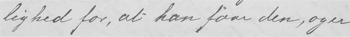lighed for, at han faar den, og er
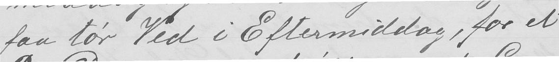faa tør Ved i Eftermiddag, for et
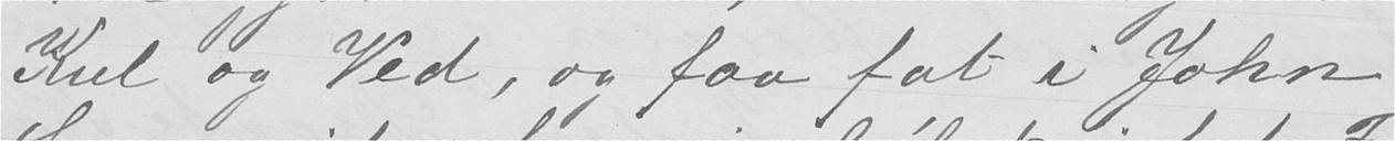Kul og Ved, og faa fat i John
